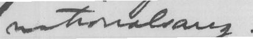nationalsang.
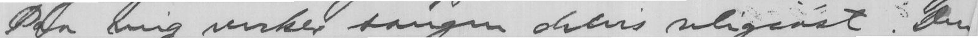Paa mig virker sangen Deres religiøst. Den
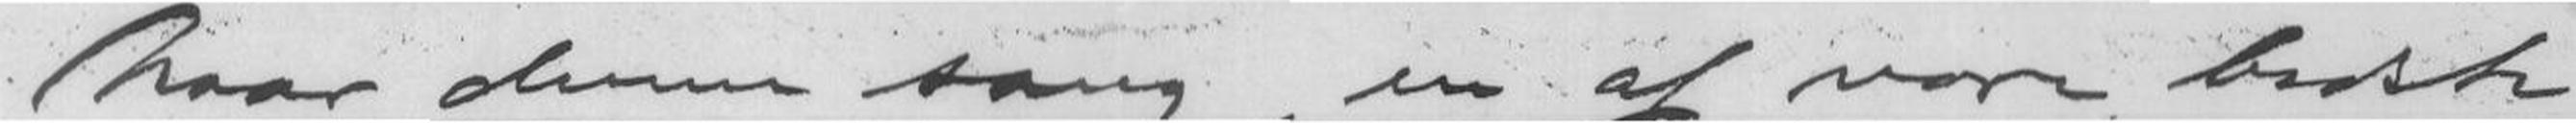Naar deres sang, en af vore bedste,
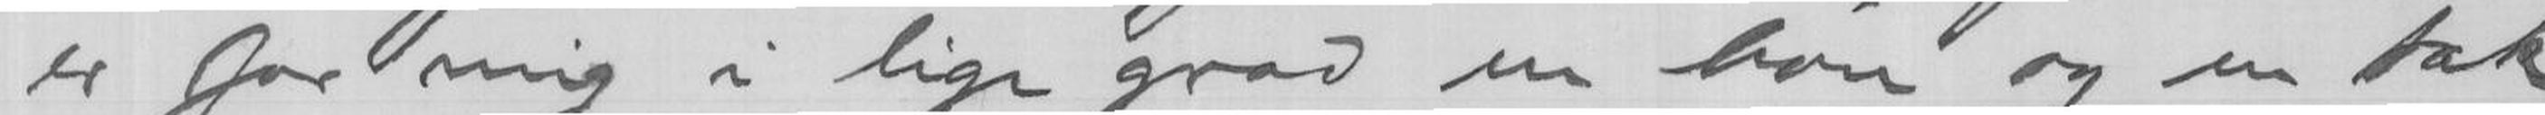er for mig i lige grad en bøn og en tak.
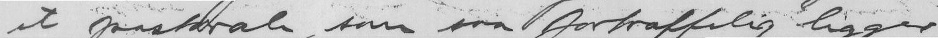et pastorale, som saa fortræffelig ligger
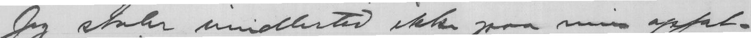Jeg stoler imidlertid ikke paa min opfat-
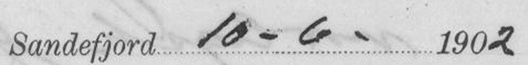Sandefjord 10 - 6 - 1902
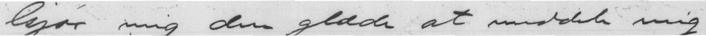Gjør mig den glæde at meddele mig,
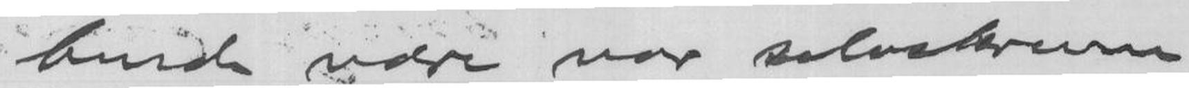burde være vor selvskrevne
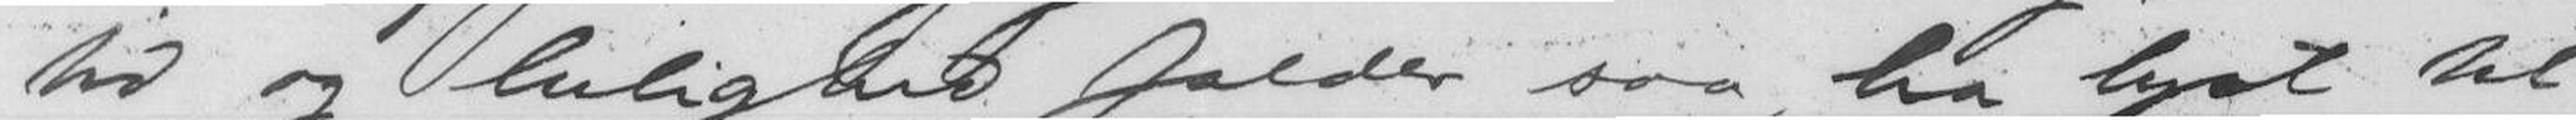tid og leilighed falder saa, ha lyst til
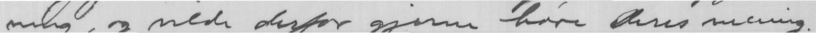ning, og vilde derfor gjærne høre Deres mening.
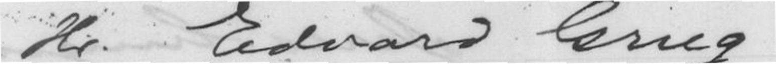Hr. Edvard Grieg
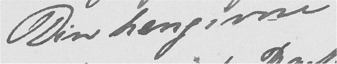Din hengivne
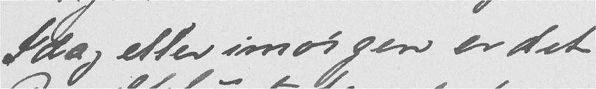Idag eller imorgen er det
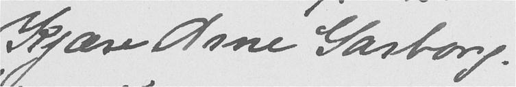Kjære Arne Garborg
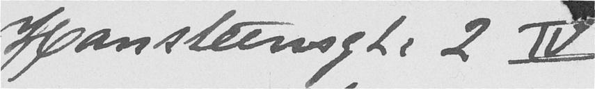Hansteensgt. 2 IV
